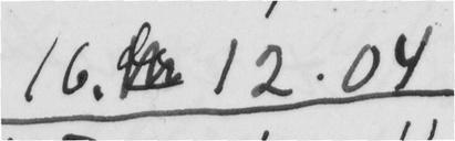16. 12.04
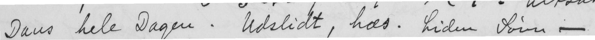Dans hele Dagen. Udslidt, hæs. Liden Søvn -
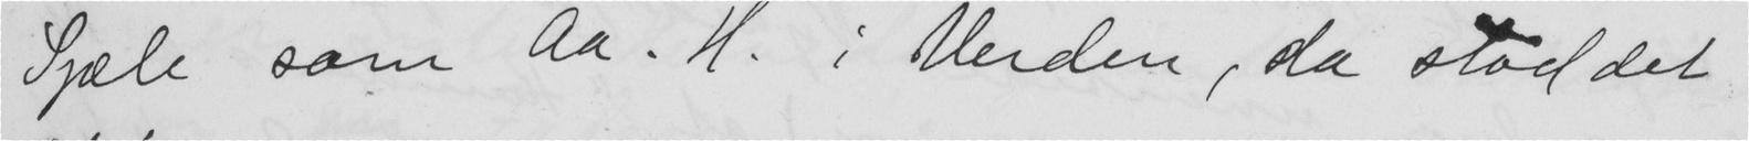Sjæle som Aa. H. i Verden, da stod det
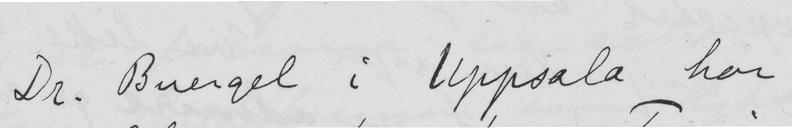Dr. Buergel i Uppsala har
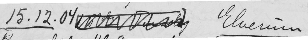15.12 04  Elverum
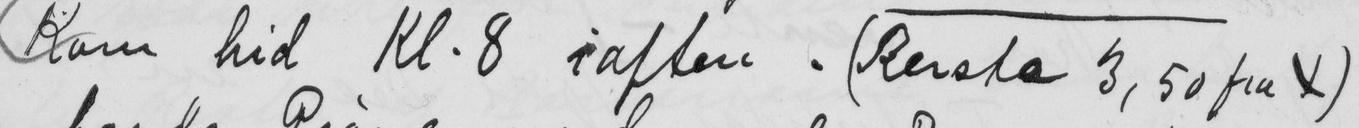Kom hid Kl. 8 iaften. (Reiste 3, 50 fra X)
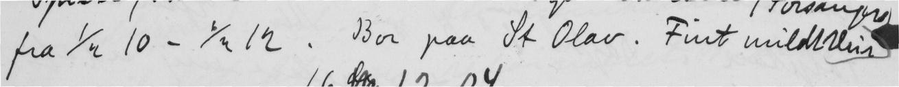fra 1/2 10 - 1/2 12. Bor paa St Olav. Fint mildtveir
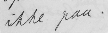ikke paa.
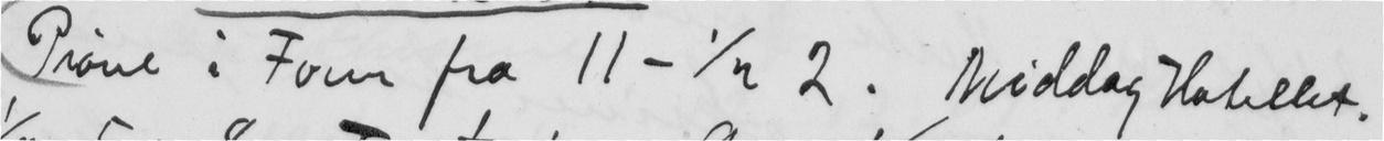Prøve i Form fra 11 - 1/2 2. Middag Hotellet.
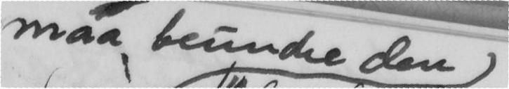maa beundre den
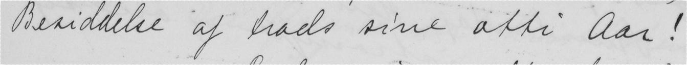Besiddelse af trods sine otti Aar!
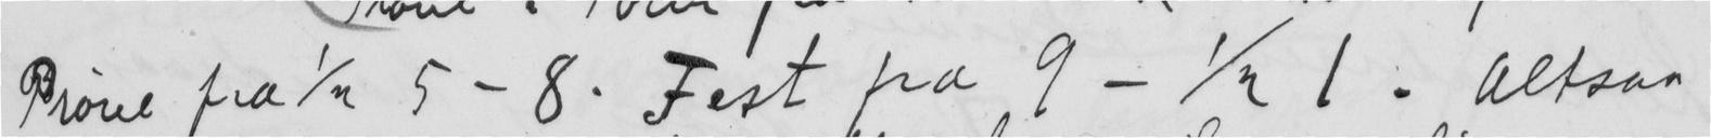Prøve fra 1/2 5 - 8. Fest fra 9 - 1½ 1. Altsaa
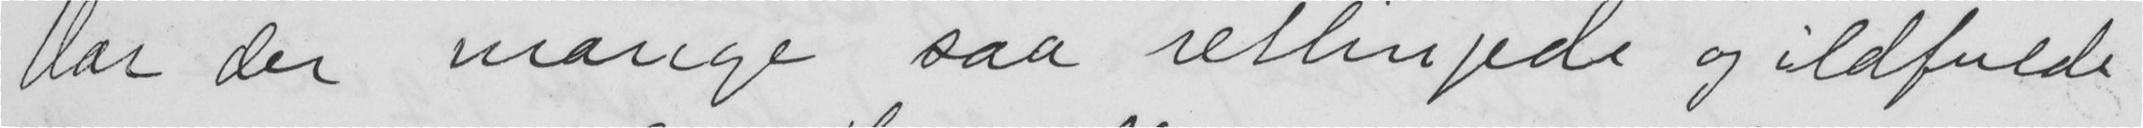Var der mange saa retlinjede og ildfulde
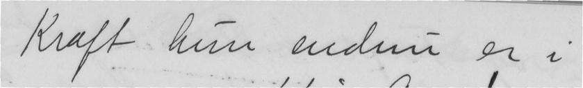Kraft hun endnu er i
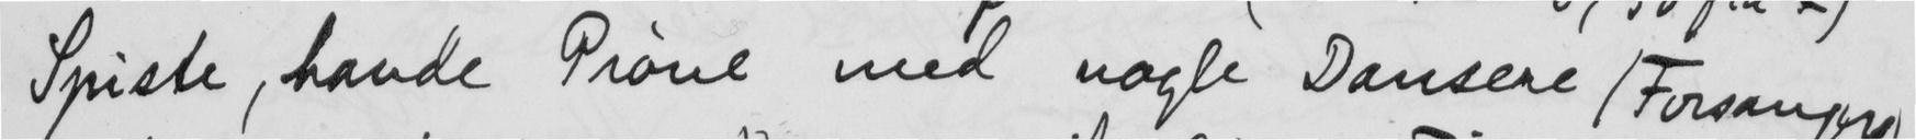Spiste, havde Prøve med nogle Dansere (Forsanger
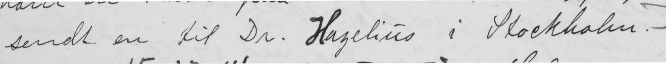sendt en til Dr. Hagelius i Stockholm.
